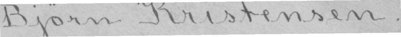Bjørn Kristensen.
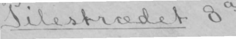Pilestrædet 8a
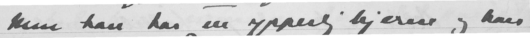Kun han har en ypperlig hjerne og han
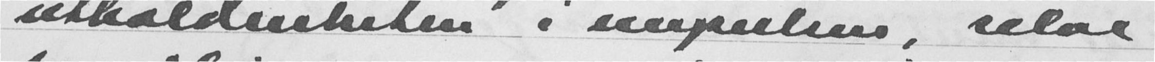utholdenheten i impulsen, selve
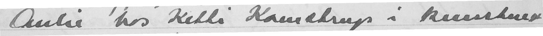Aulie hos Kitti Kamstrup i kunstner-
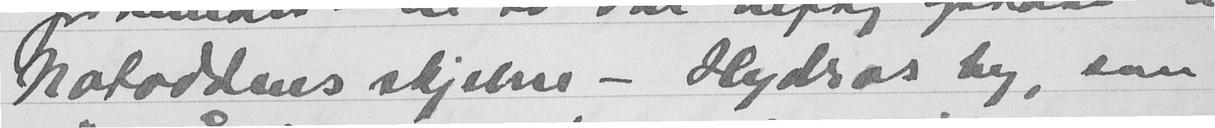Notoddens skjebe - Hydras by, som
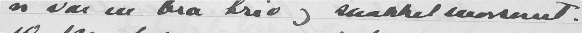vi var en bra trio og snakket morsomt.
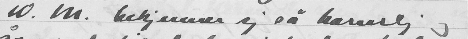W. M. bekjenner sig så barnlig
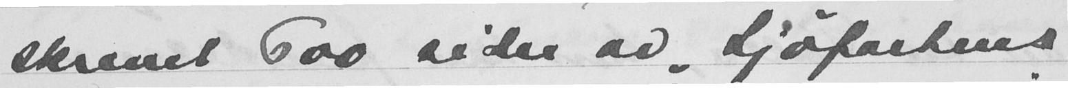skrevet 500 sider av Sjøfartens
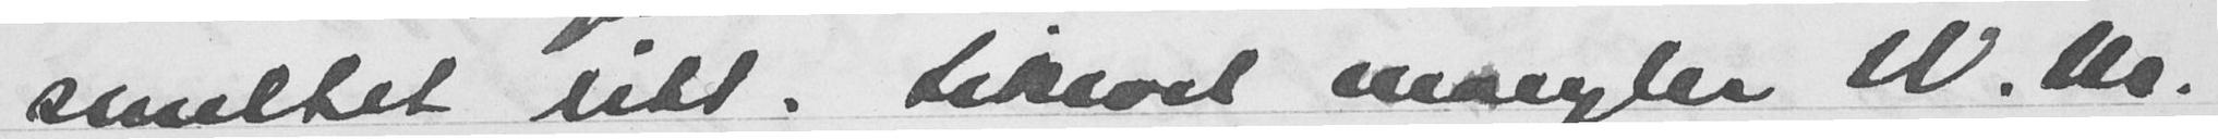smeltet litt. Likevel mangler W. M.
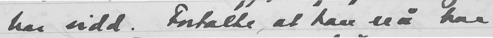har vidd. Fortalte at han nå har
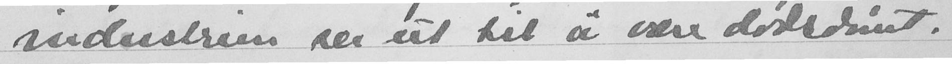industrien ser ut til å være dødsdømt.
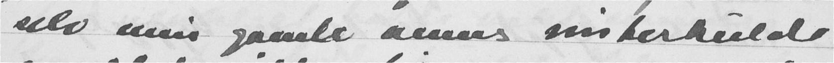selv min gamle venns vinterkulde
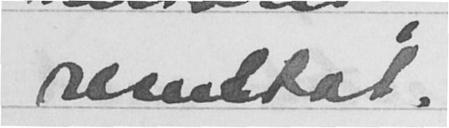resultat.
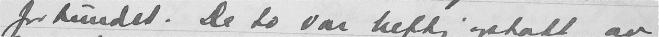forbundet. De to var heftig optatt av
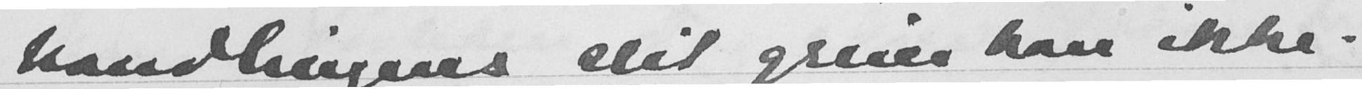handlingens slit greier han ikke.
